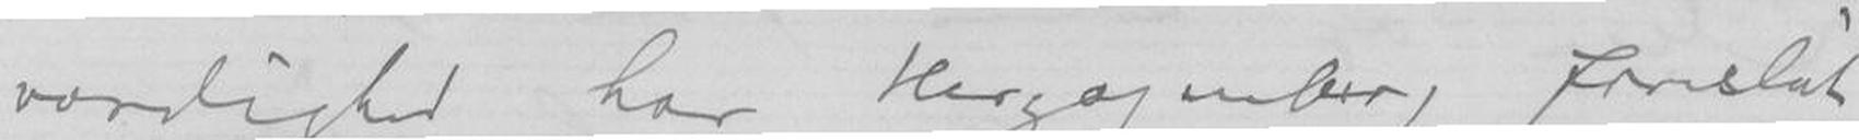værdighed har Herzogenberg foreslåt
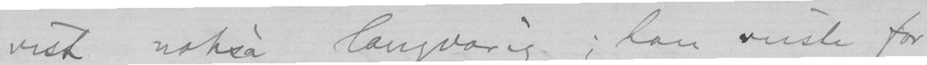vist nokså langvarig; han reiste for
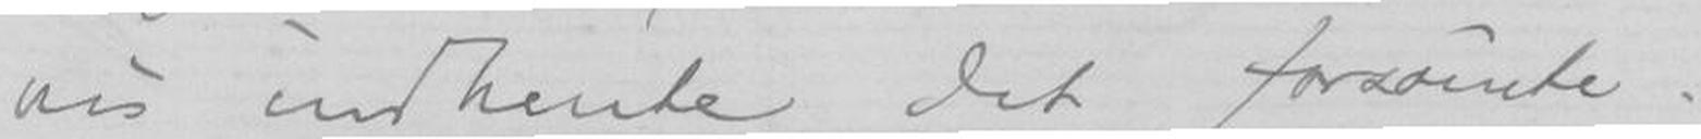vis indhente det forsømte.
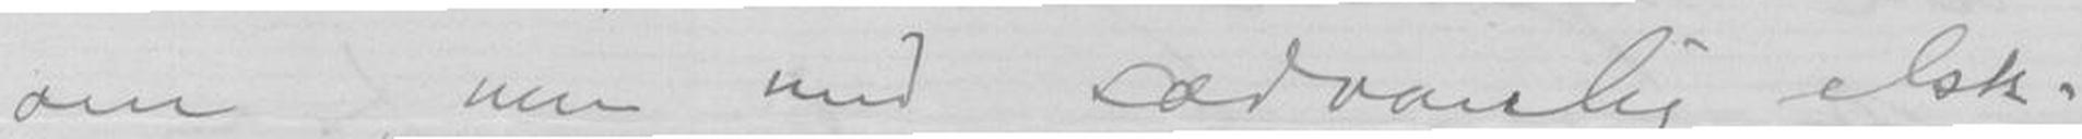om, men med sædvanlig elsk-
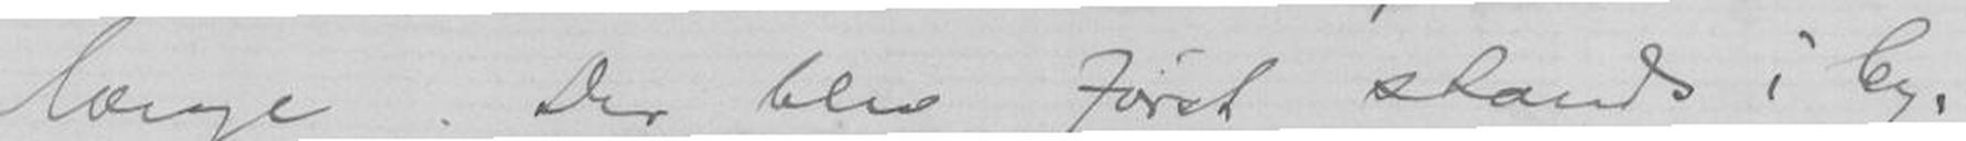længe. Der blev først stands i beg.
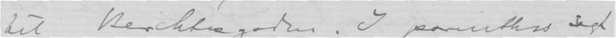til Berchtregaden. I parenthes sagt
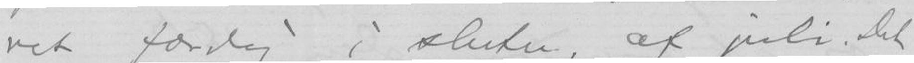ret færdig i slutten. af juli. Det
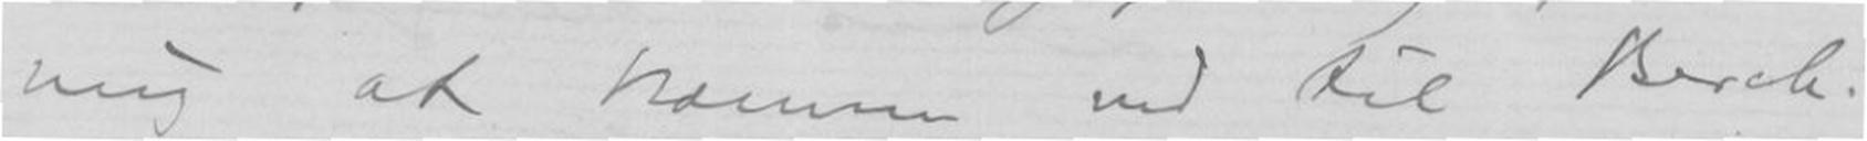mig at komme ned til Berch-
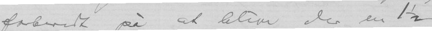forberedt på at blive der en 1½
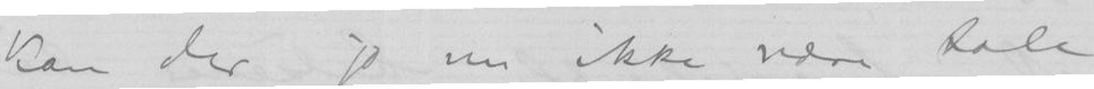kan der jo nu ikke være tale
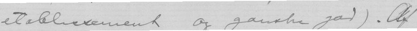eteblissement og ganske god). Af
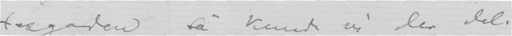tasgaden, så kunde vi der del-
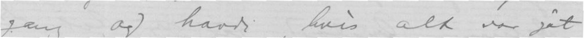gang og havde, hvis alt var gåt
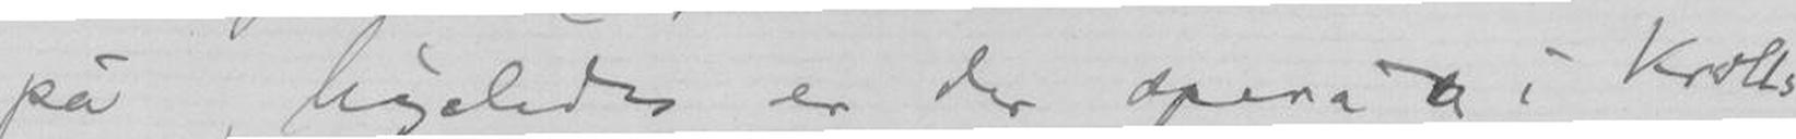på, ligeledes er der opera'n i Krolls
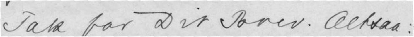Tak for dit Brev Altsaa:
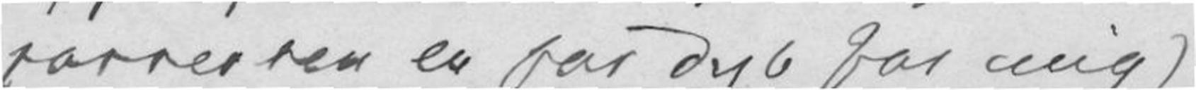forresten er for dyp for mig
